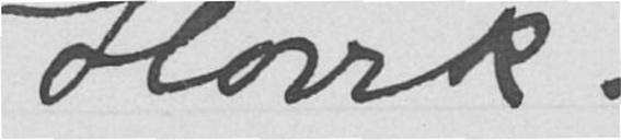Høvik
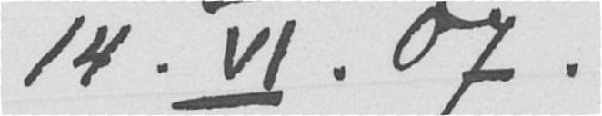14.VI.07.
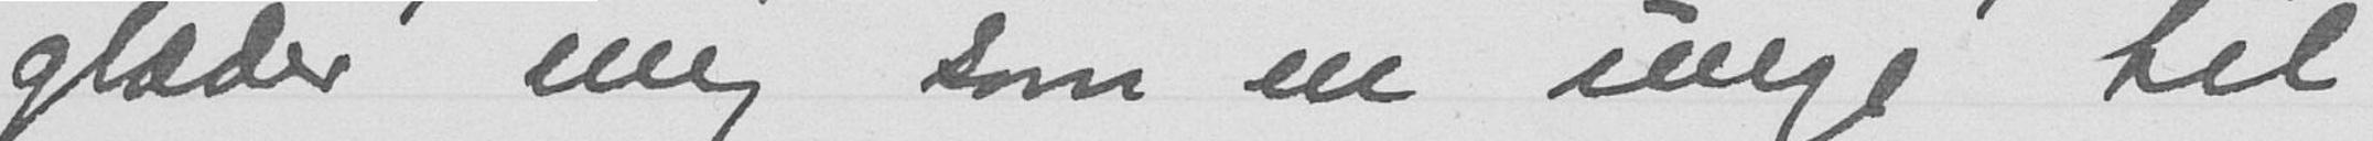glæder mig som en unge til
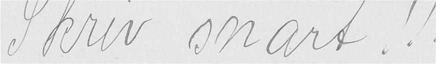Skriv snart!!!!
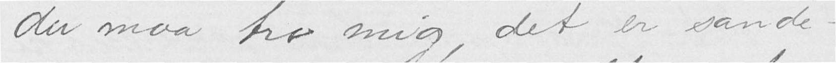du maa tro mig, det er sande-
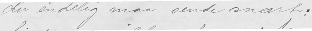du endelig maa sende snart:
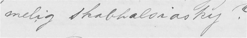melig Skabhalsiasky?
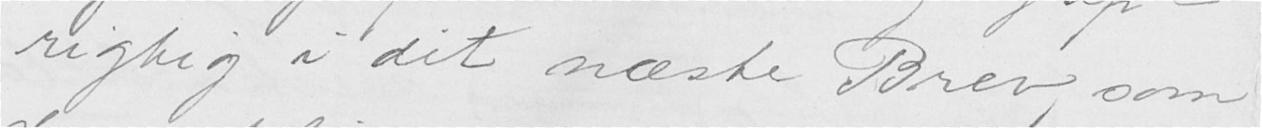riktig i dit næste Brev, som
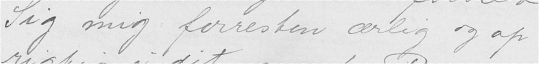Sig mig forresten ærlig og op-
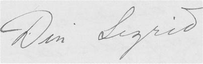Din Sigrid.
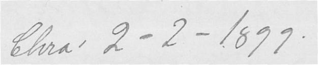Chra 2-2-1899.
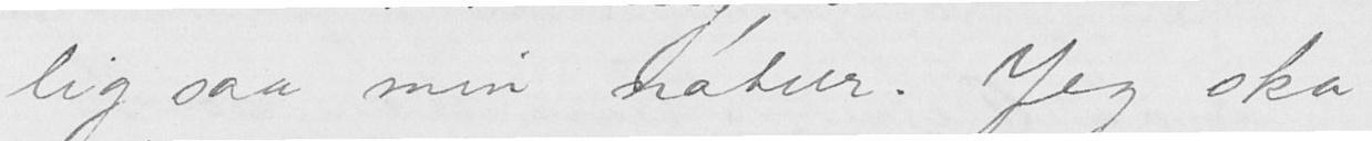lig saa min natur. Jeg ska-
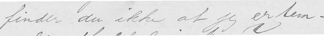finder du ikke at jeg er tem-
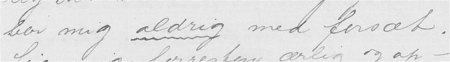ber mig aldrig med forsæt.
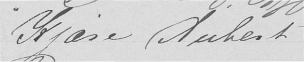Kjære Aubert
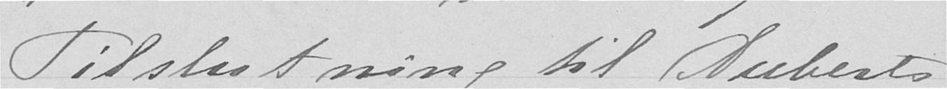Tilslutning til Auberts
